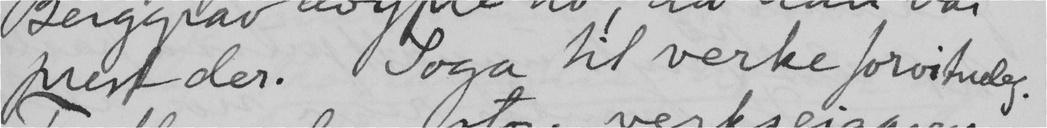prest der. Soga til verke forvitneleg.
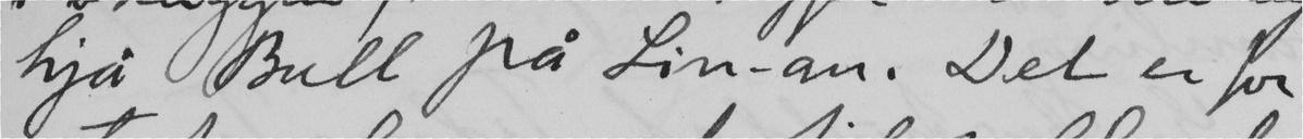hjå Bull på Lin-an. Det er for
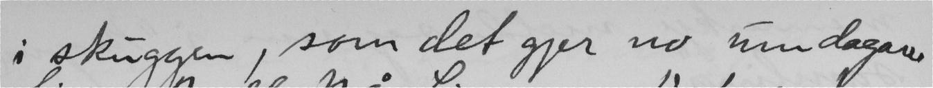i skuggen, som det gjer no umdagane
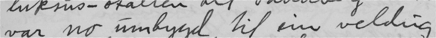var no umbygd til ein veldug
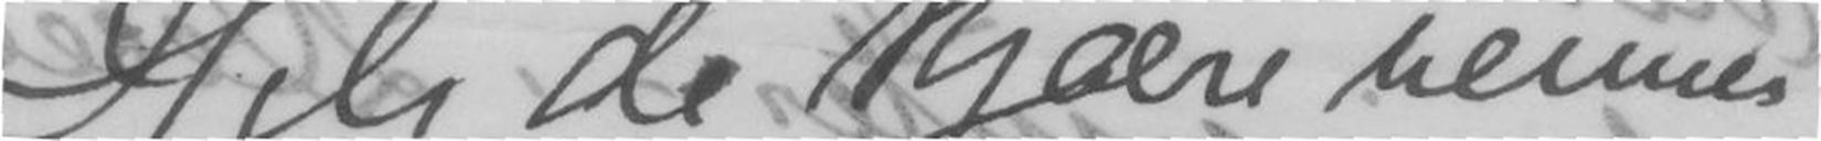Hils de kjære venner
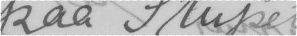paa Stupet.
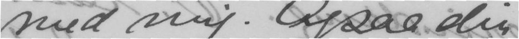med mig. Ogsaa din
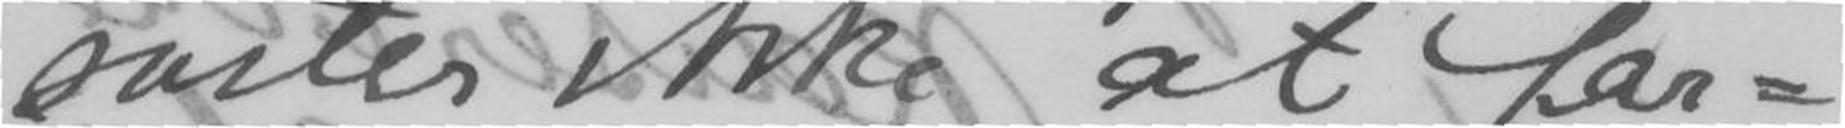søster ikke at for=
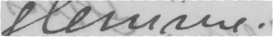glemme.
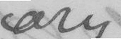Bjørn
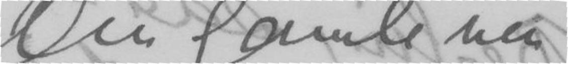Din gamle ven
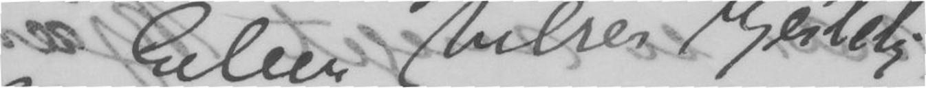og Eilev hilser hjertelig
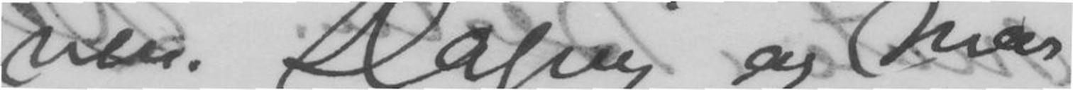ven. Dagny og Mor
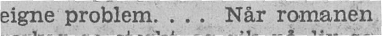eigne problem.... Når romanen
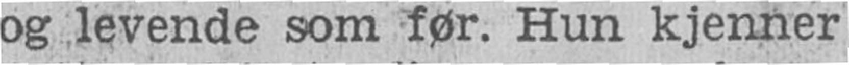og levende som før. Hun kjenner
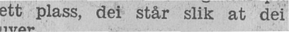rett plass, dei står slik at dei
